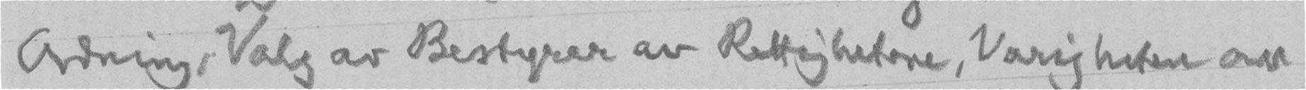Ordning, Valg av Bestyrer av Rettighetene, Varigheten av
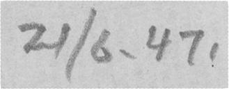21/6. 47.
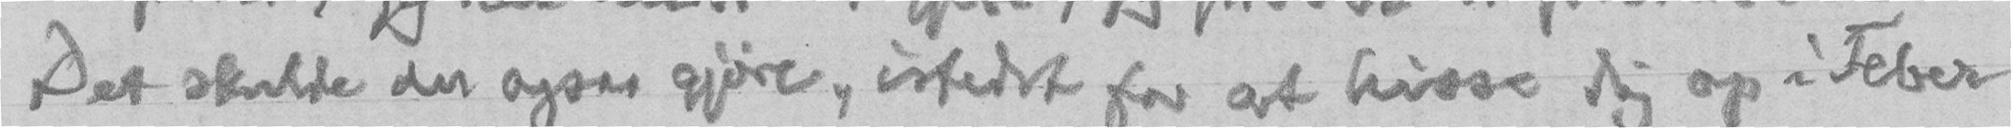Det skulde du ogsaa gjøre, istedet for at hisse dig op i Feber
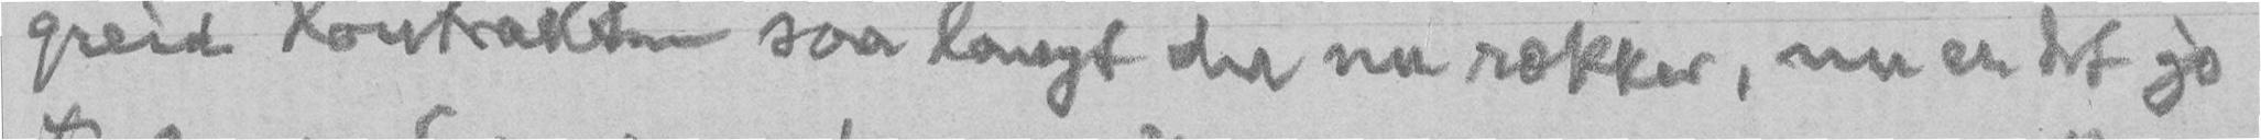greid Kontrakten saa langt den nu rækker, nu er det jo
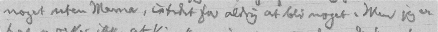noget uten Mama, istedet for aldrig at bli noget. Men jeg er
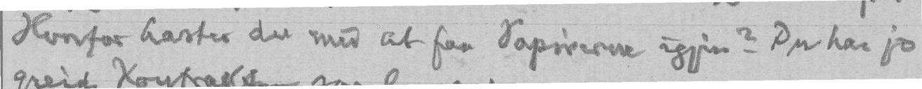Hvorfor haster du med at faa Papirerne igjen? Du har jo
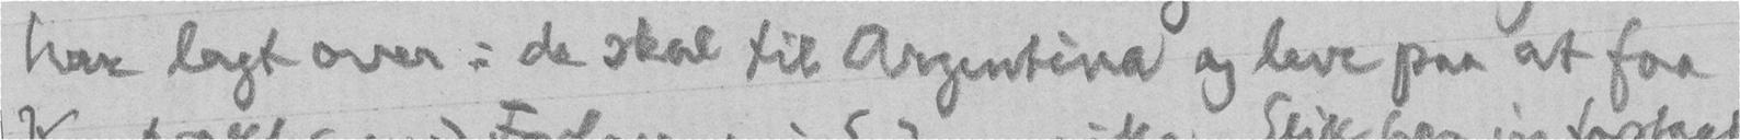har lagt over: de skal til Argentina og leve paa at faa
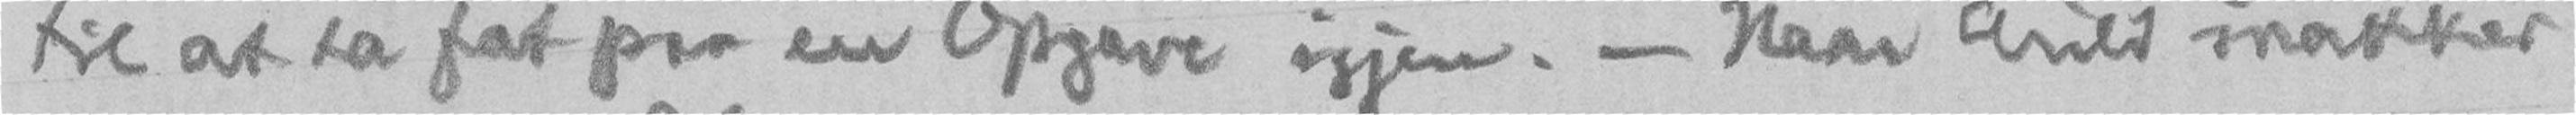til at ta fat paa en Opgave igjen. - Naar Arild snakker
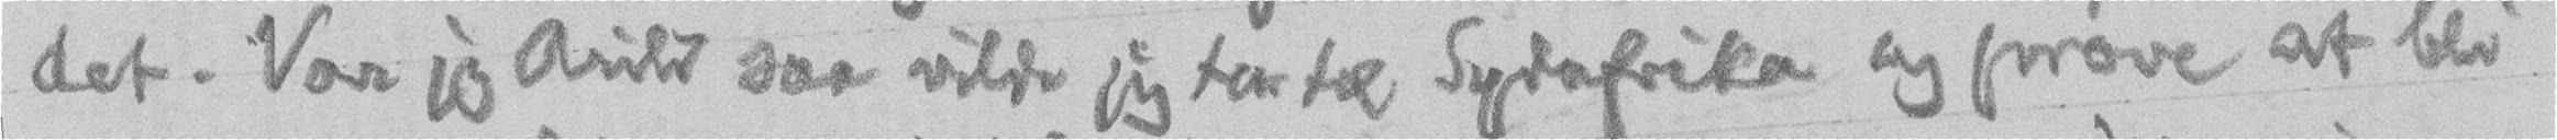det. Var jeg Arild saa vilde jeg ta til Sydafrika og prøve at bli
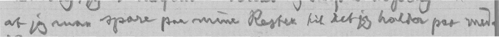at jeg maa spare paa mine Rester til det jeg holder paa med.
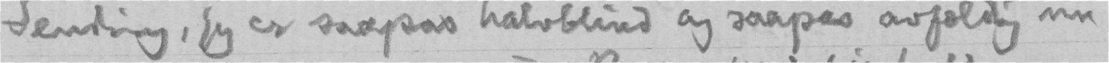Sending, jeg er saapas halvblind og saapas avfældig nu
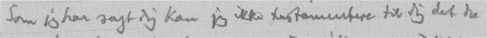Som jeg har sagt dig kan jeg ikke testamentere til dig det du
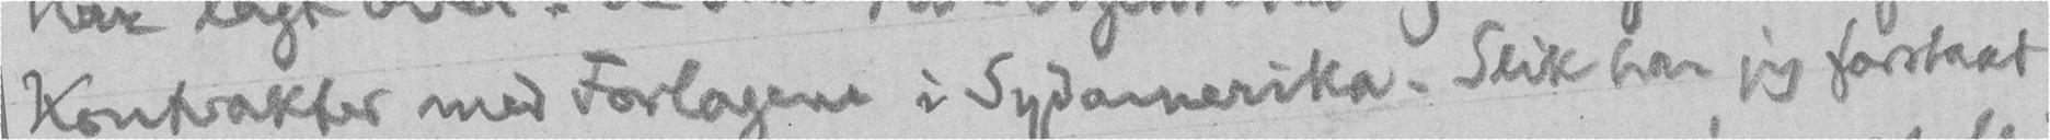Kontrakter med Forlagene i Sydamerika. Slik har jeg forstaat
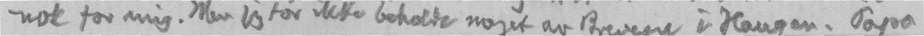nok for mig. Men jeg tør ikke beholde noget av Brevene i Haugen. Papa
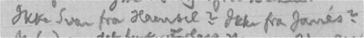Ikke Svar fra Haensel? Ikke fra Janés?
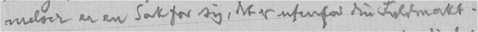melser er en Sak for sig, det er utenfor din Fuldmakt.
